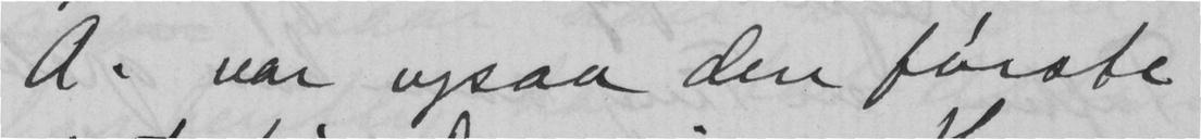A. var ogsaa den første
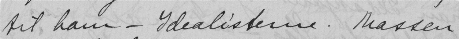til ham - Idealisterne. Massen
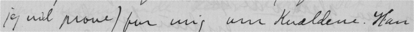jeg vil prøve) for mig om Kvældene. Han
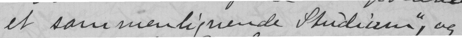et sammenlignende Studium", og
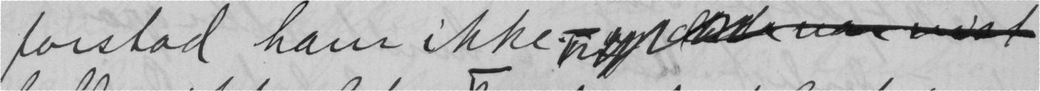forstod ham ikke ogg.
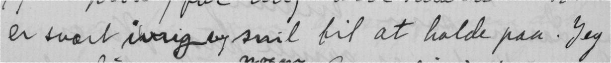er svært  snil til at holde paa. Jeg
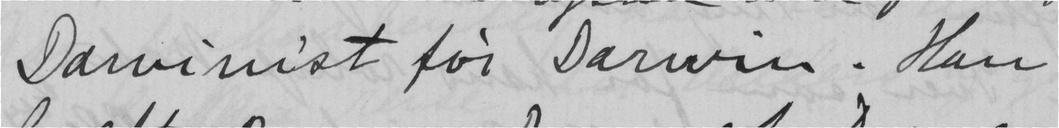Darvinist før Darwin. Han
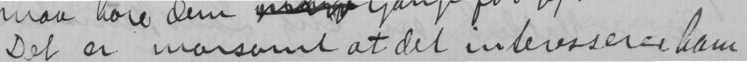Det er morsomt at det interesserer ham
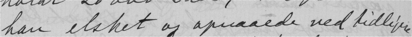han elsket og opnaaede ved tidligere
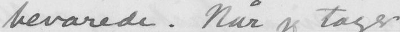bevarede. Når jeg tager
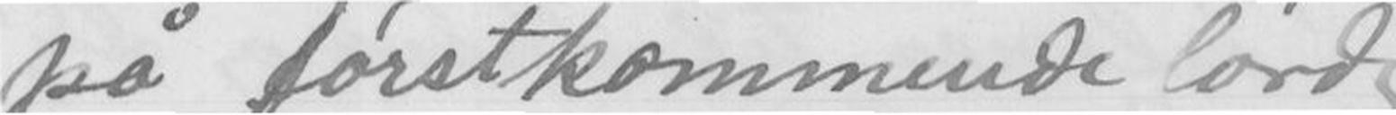på førstkommende lørdag.
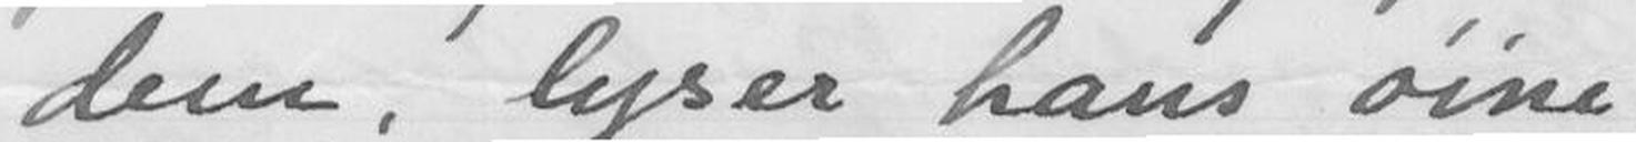dem, lyser hans øine
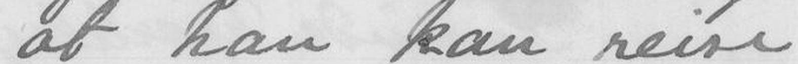at han kan reise
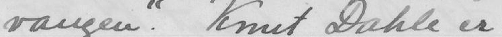vangen." Knut Dahle er
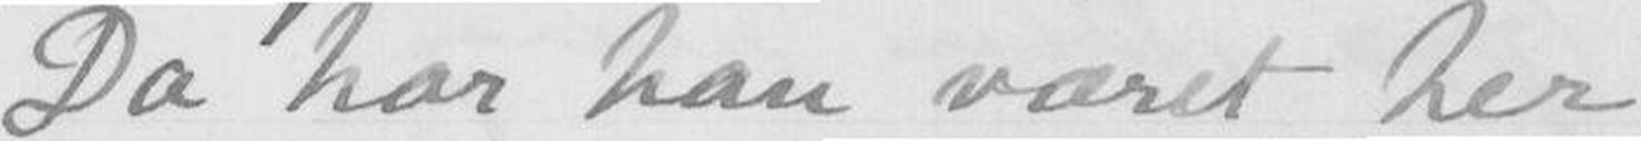Da har han været her
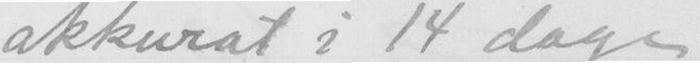akkurat i 14 dage.
